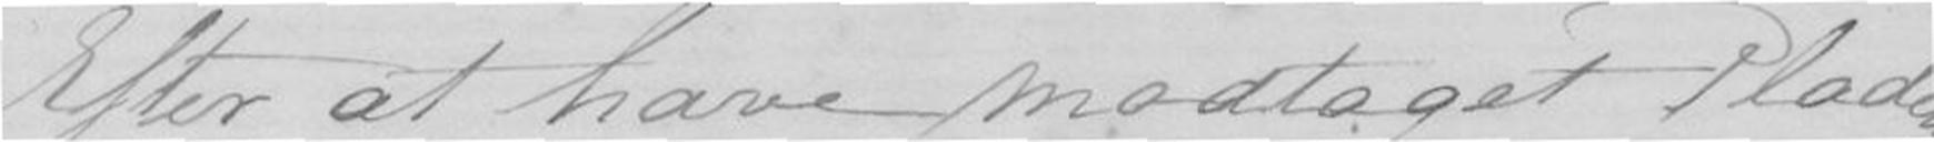Efter at have medtaget Pladsen
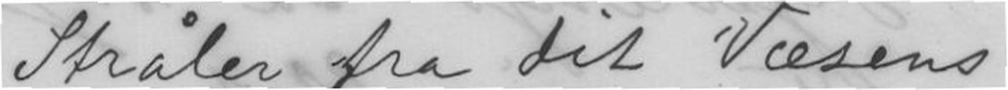Stråler fra det Væsens
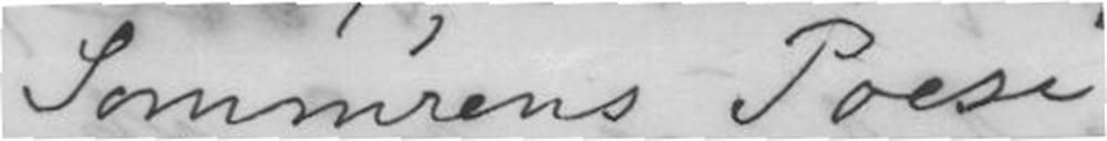Sommerens Poesi
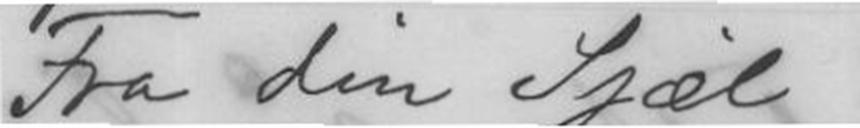Fra din Sjæl
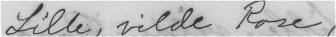Lille, vilde Rose,
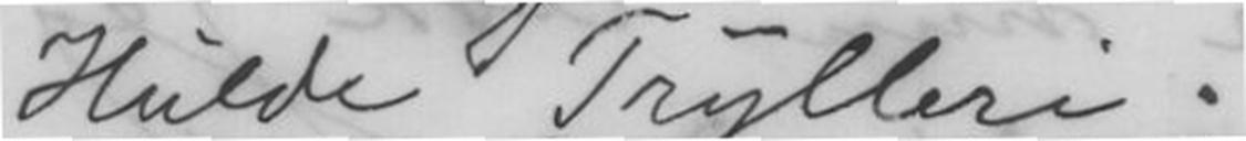Hulde Trylleri.
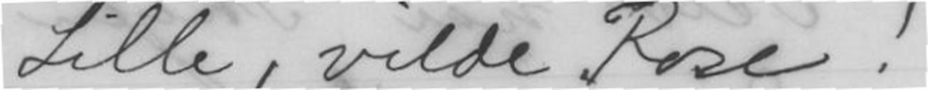Lille, vilde Rose!
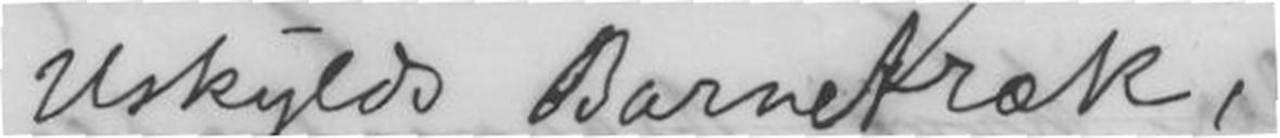Uskylds Barnetræk,
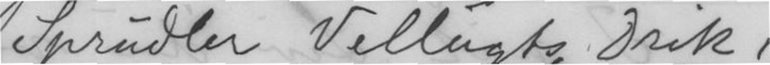Sprudler Vellugts Drik,
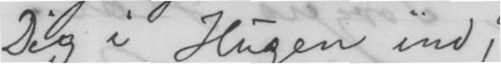Dig i Hugen ind,
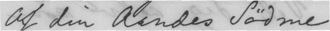Af din Aandes Sødme
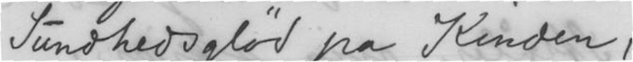Sundhedsglød på Kinden,
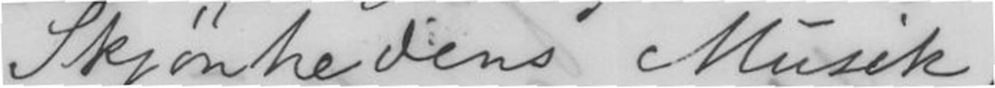Skjønhedens Musik,
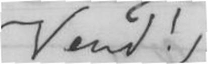(Vend!)
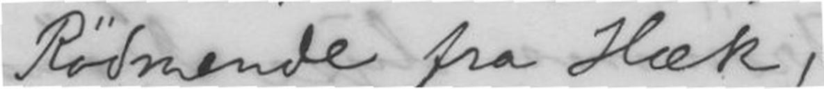Rødmende fra Hæk,
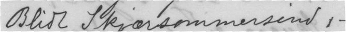Blidt Skjærsommersind,
